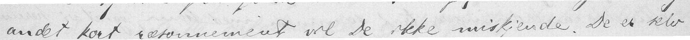andet kort ræsonnement, vil De ikke miskjende. De er selv
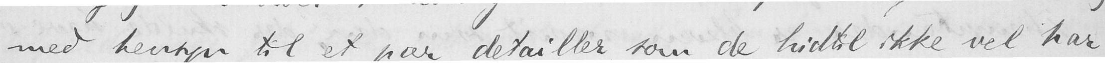med hensyn til et par detailler, som de hidtil ikke vel har
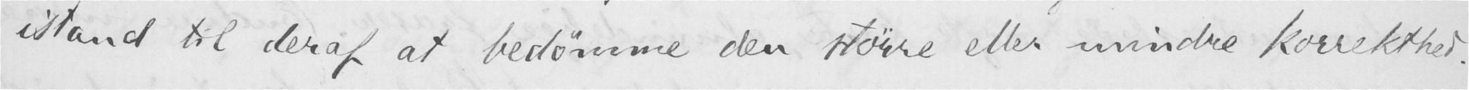istand til deraf at bedømme den større eller mindre korrekthed.
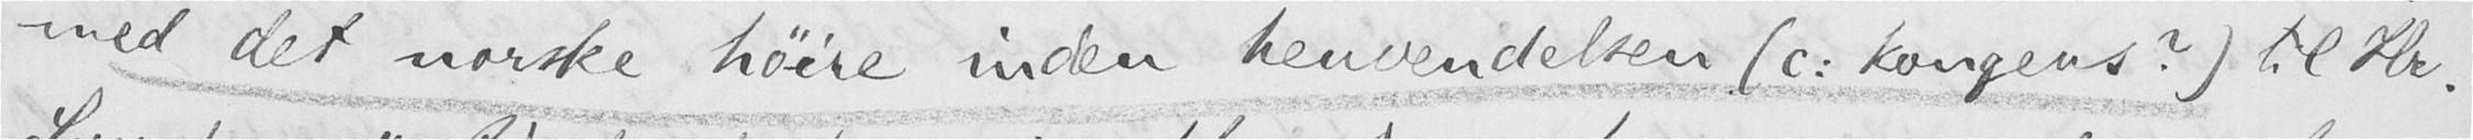med det norske høire inden henvendelsen (c: kongens?) til Hr.
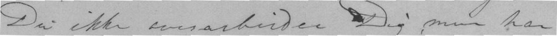Du ikke overarbeider Dig, men har
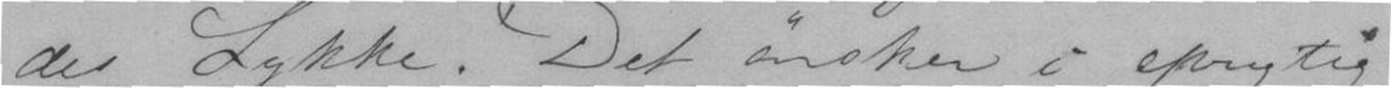des Lykke. Det ønsker i oprigtig
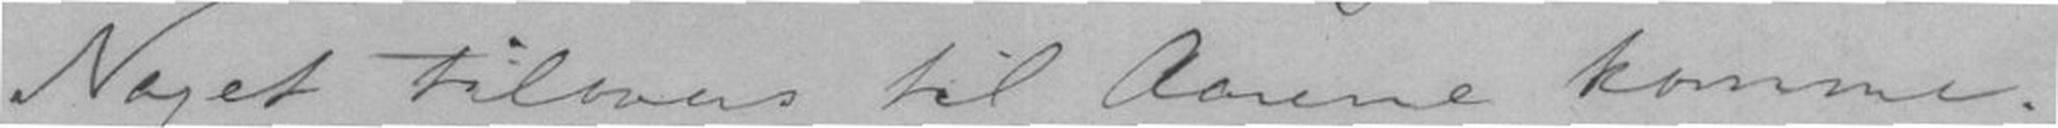Noget tilovers til Aarene komme.
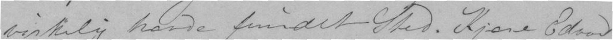virkelig havde fundet Sted. Kjære Edvard
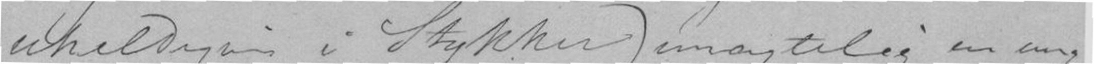uheldigvis i Stykker) unægtelig en ung
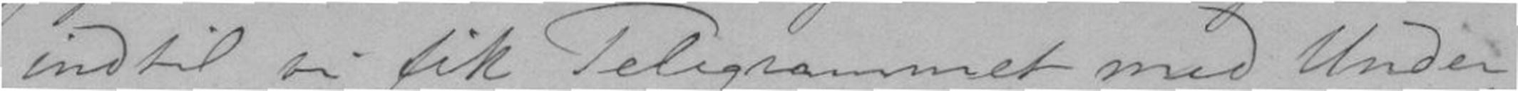indtil vi fik Telegrammet med Under-
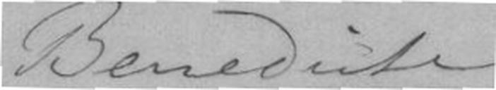Benedicte.
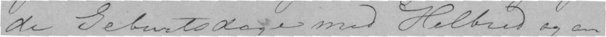de Geburtsdage med Helbred og an-
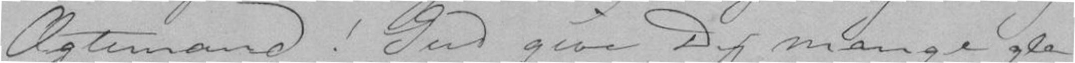Ægtemand! Gud give Dig mange gla-
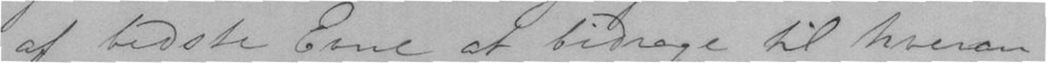af bedste Evne at bidrage til hveran-
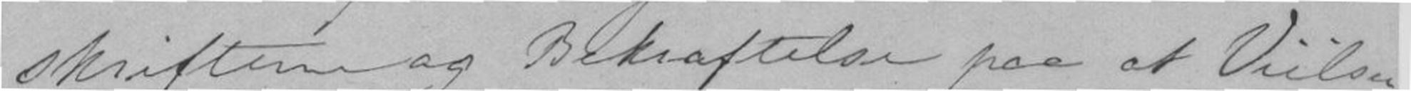skriften og Bekræftelse paa at Vielsen
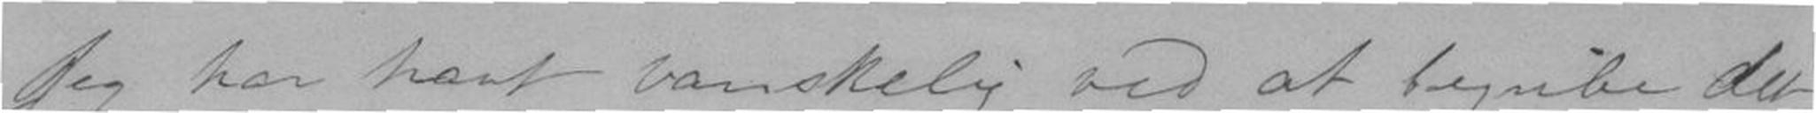Jeg har havt vanskelig ved at begribe det
